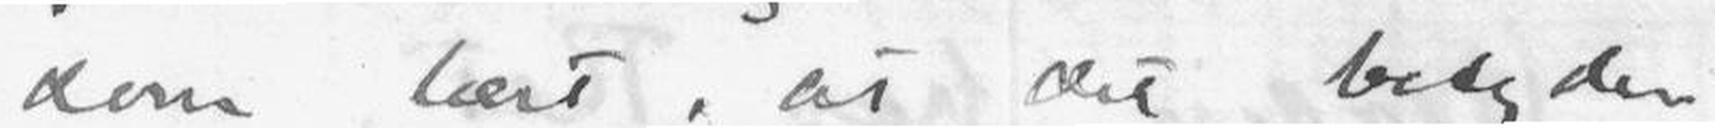dom læst, at det betyder
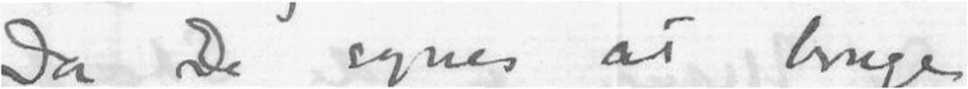Da De synes at bruge
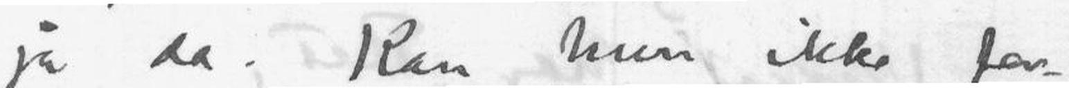så da. Kan hun ikke for-
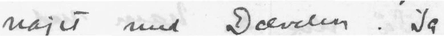nojet med Dævelen. Ja
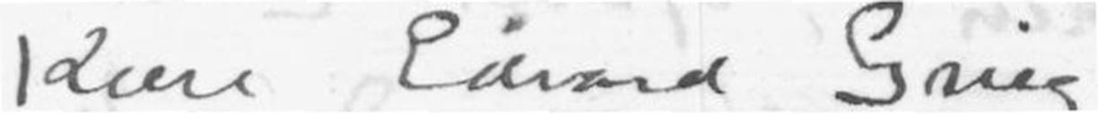Kære Edvard Grieg!
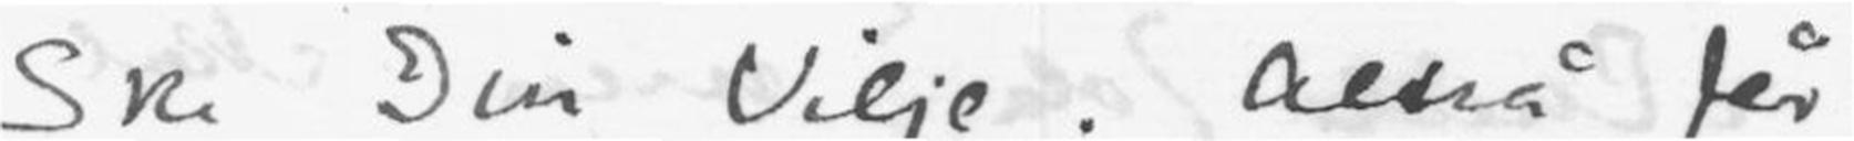Ske Din Vilje. Altså får
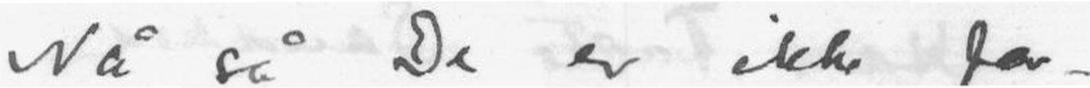Nå så De er ikke for
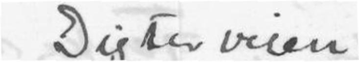Digtervisen.
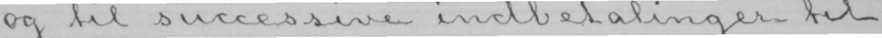og til successive indbetalinger til
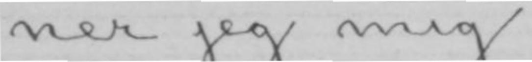ner jeg mig
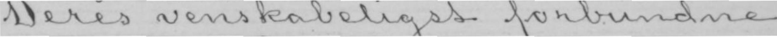Deres venskabeligst forbundne
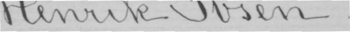Henrik Ibsen.
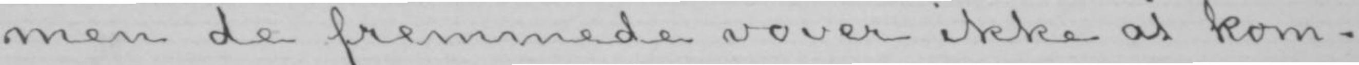men de fremmede vover ikke at kom-
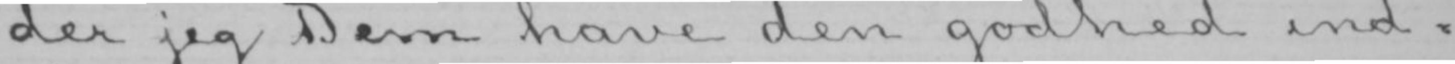der jeg Dem have den godhed ind-
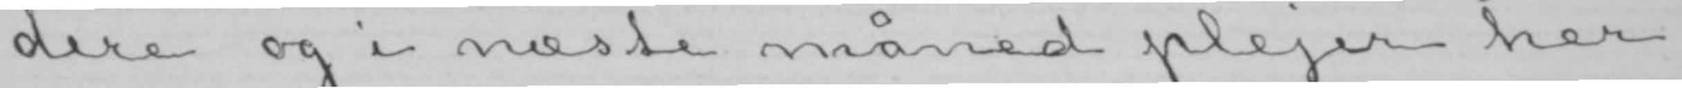dere og i næste måned plejer her
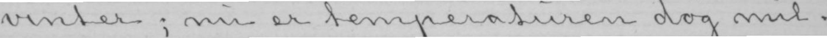vinter; nu er temperaturen dog mil-
